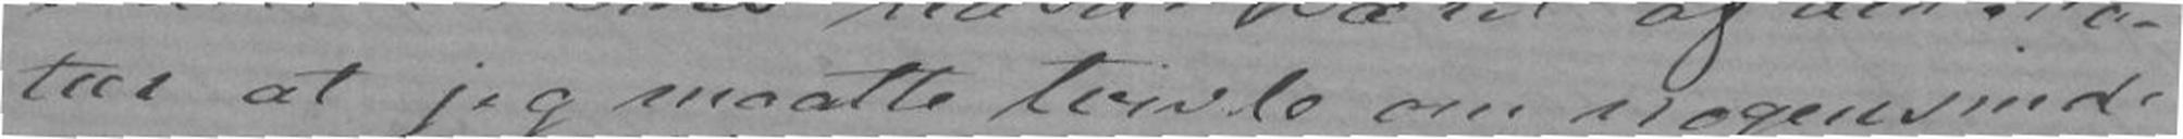tur at jeg maatte tvivle om nogensinde
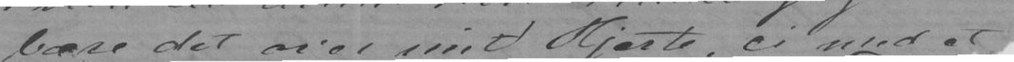bære det over mit Hjerte, ei med et
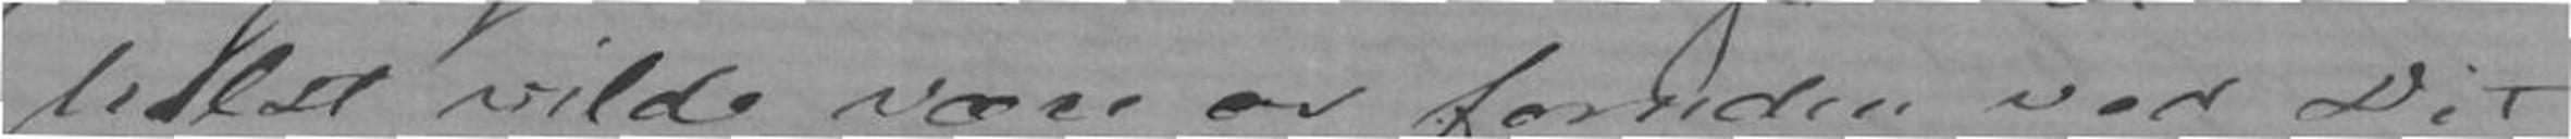helst vilde være os foruden ved Dit
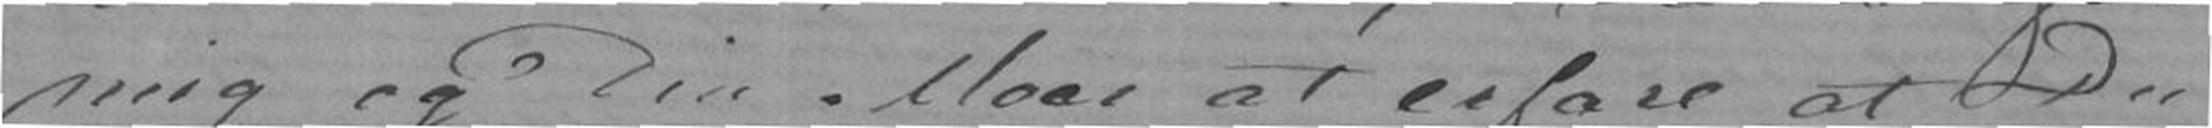mig og Din Moer at erfare at Du
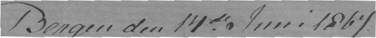Bergen den 14de Juni 1867.
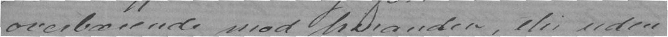overbærende med hinanden, thi uden
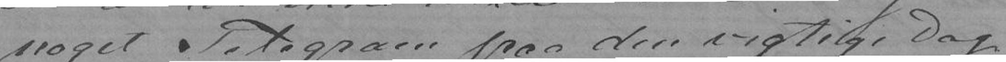noget Telegram paa den vigtige Dag.
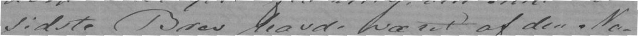sidste Brev havde været af den Na-
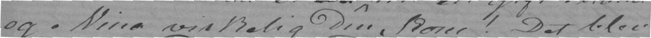og Nina virkelig Din kone! Det blev
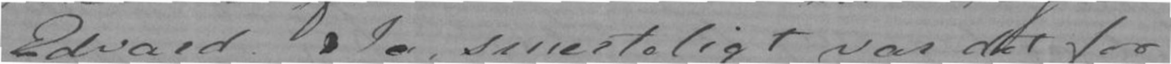Edvard. Ja, smerteligt var det for
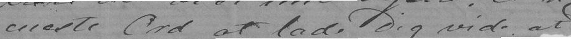eneste Ord at lade Dig vide at
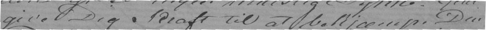give Dig Kraft til at bekjæmpe Din
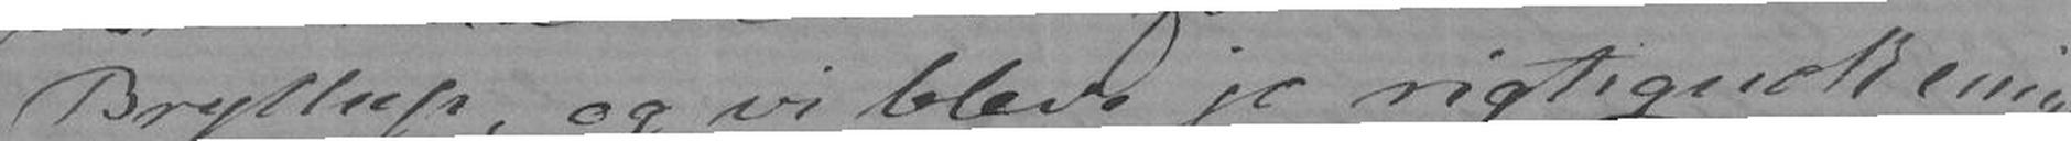Bryllup, og vi bleve jo rigtignok enige
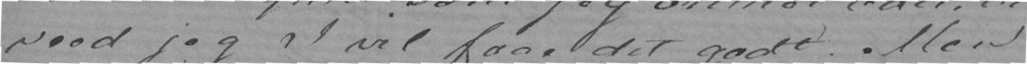veed jeg I vil faae det godt. Men
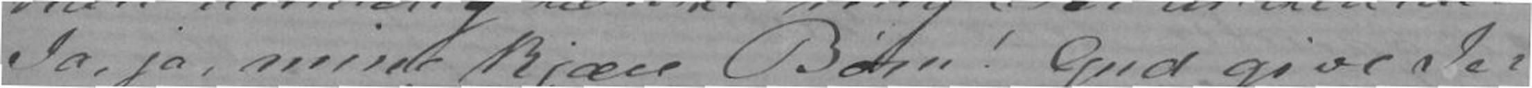Ja, ja, mine kjære Børn! Gud give Jer
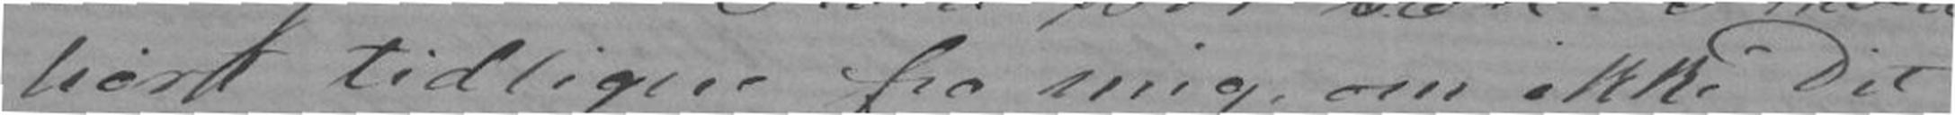hørt tidligere fra mig, om ikke Dit
